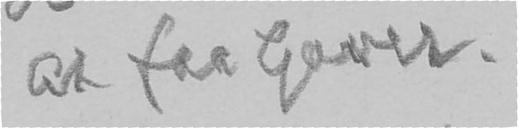at faa Gaver.
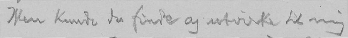Men kunde du finde og utvirke til mig
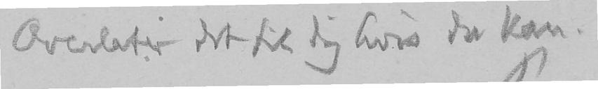Overlater det til dig hvis du kan.
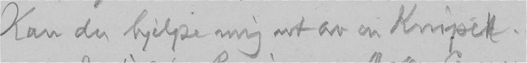Kan du hjelpe mig ut av en Knipee.
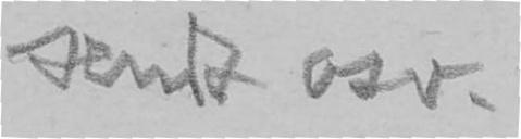sendt osv.
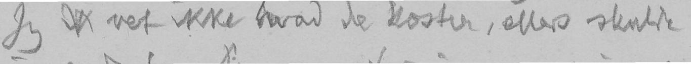Jeg ik vet ikke hvad de koster, ellers skulde
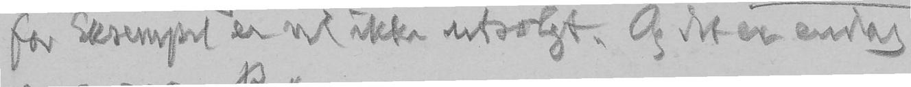for Eksempel er vel ikke utsolgt. Og det er endog
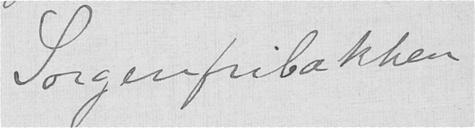Sorgenfribakken
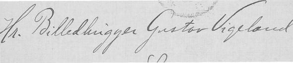Hr. Billedhugger Gustav Vigeland
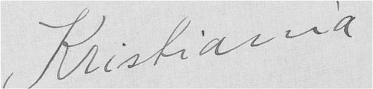Kristiania
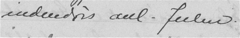indendørs anl. Julen.
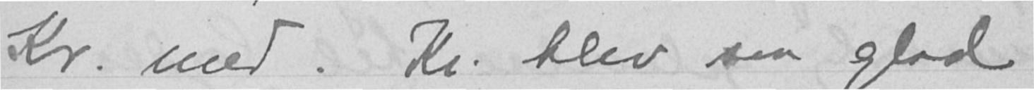Kr. med. Kr. blev saa glad
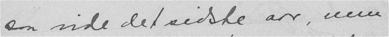saa vide det sidste aar, men
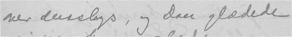over denslags, og Don glædede
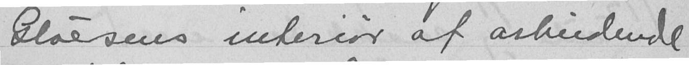Gløersens interiør af arbeidende
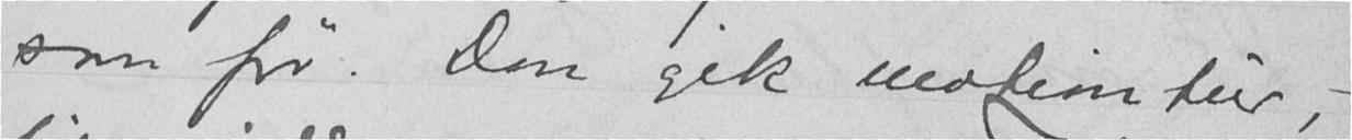som før. Don gik motiontur, -
